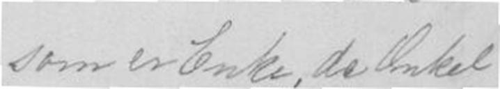som er Enke, de Onkel
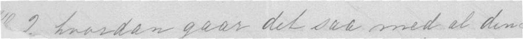hvordan gaar det saa med al den
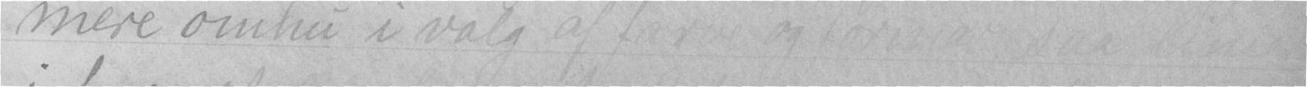mere omhu i valg at farve og former, saa
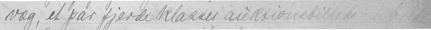væg, et par fjerde klasses auktionsbillede i brede
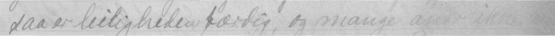saa er leiligheden færdig, og mange aner ikke en-
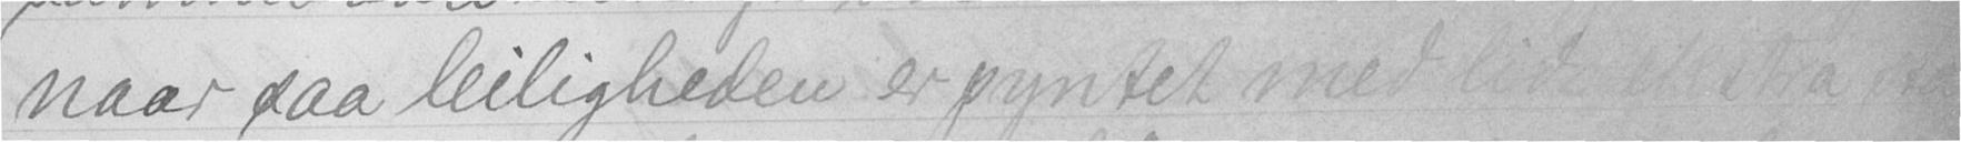naar saa leiligheden er pyntet med lidt ekstra
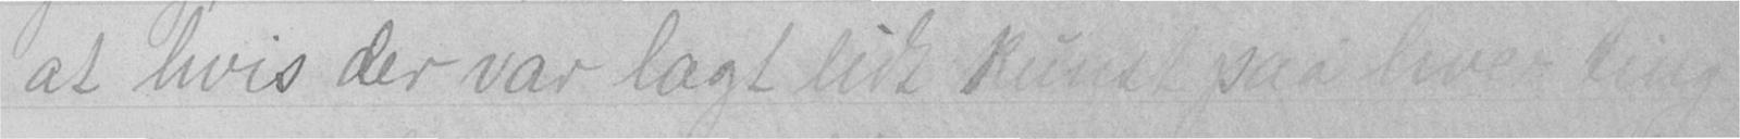at hvis der var lagt lidt kunst paa hver ting
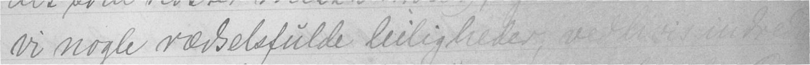vi nogle rædselsfulde leiligheder, ved hvis indredning
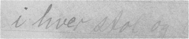i hver stol og hvert bord kunne glæde øiet,
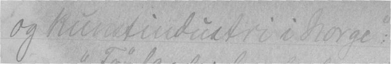og kunstindustri i Norge":
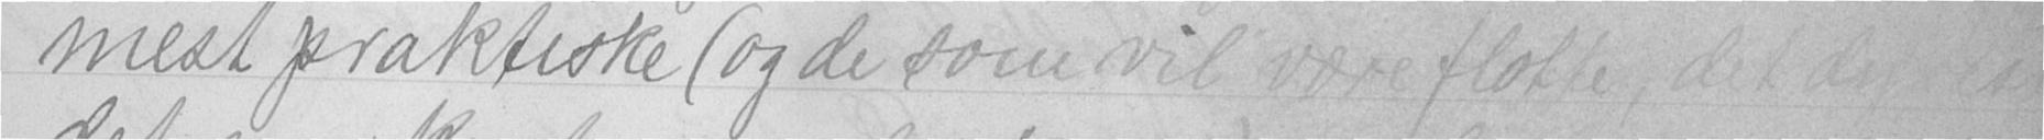mest praktiske (og de som vil være flotte, det
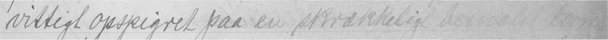vittigt opspigret paa en skrækkelig bemalet lærret-
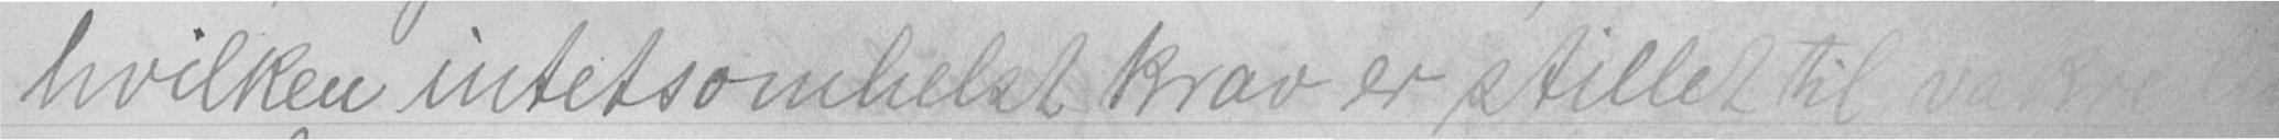hvilken intetsomhelst krav er stillet til vakre
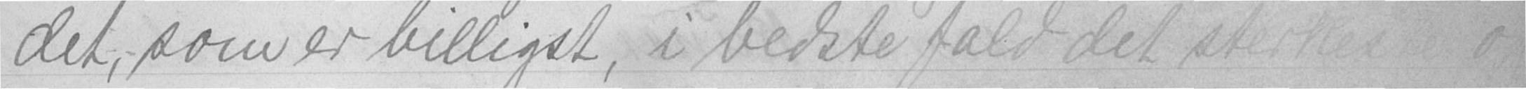det, som er billigst, i bedste fald det sterkeste og
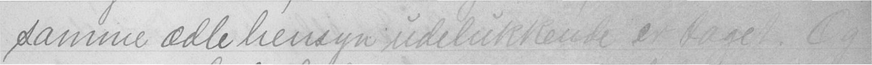samme ædle hensyn udelukkende er taget. Og
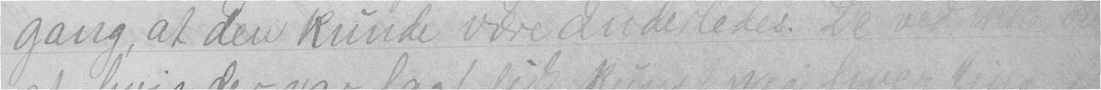gang, at den kunde være anderledes. De ved ikke
